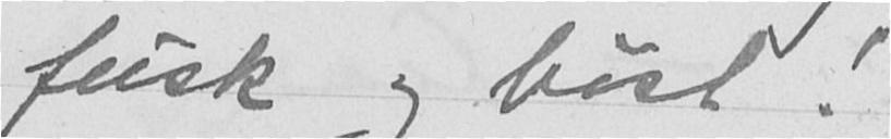fusk og høst!
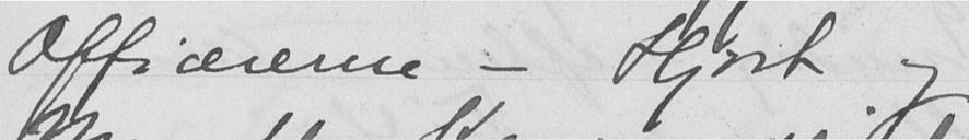Officererne - Hjort og
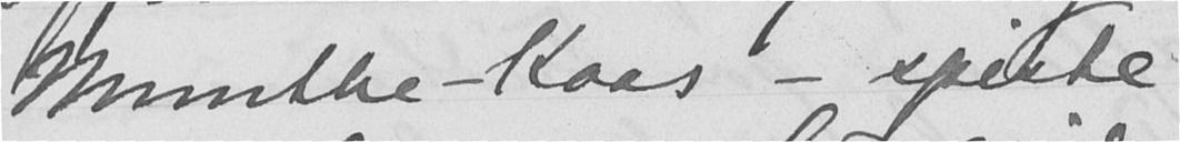Munthe-Kaas - spiste
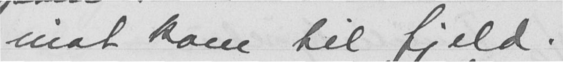inat kom til fjeld.
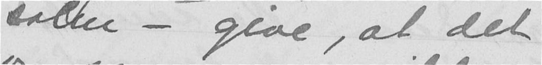solen - give, at det
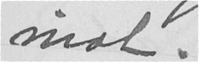inat.
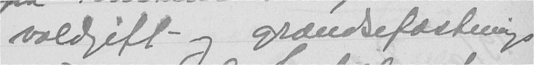voldgift- og grændsefæstnings
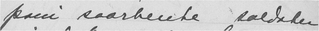pani saarbente soldater
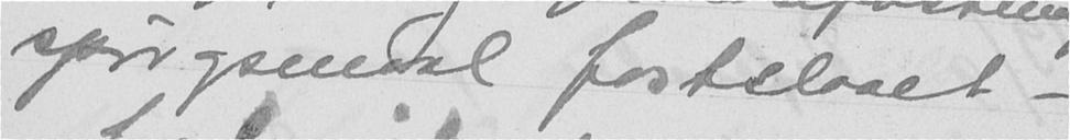spørgsmaal foreslaaet -
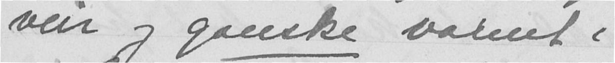veir og ganske varmt i
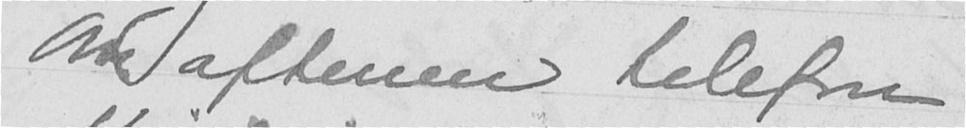Om aftenen telefon
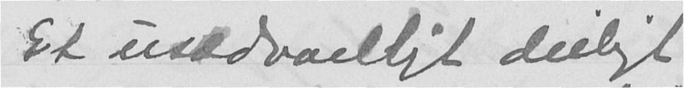Et usædvanligt deiligt
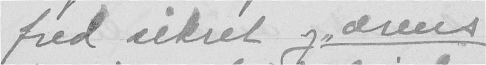fred sikret og "ærens
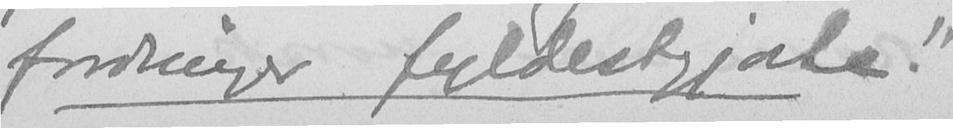fordringer fuldestgjorte."
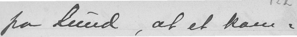fra Sund, at et kom-
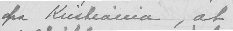fra Kristiania, at
